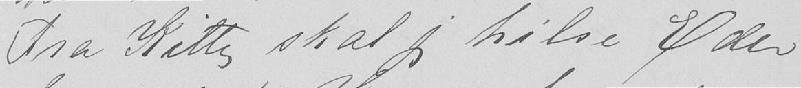Fra Kitty skal jeg hilse Eder
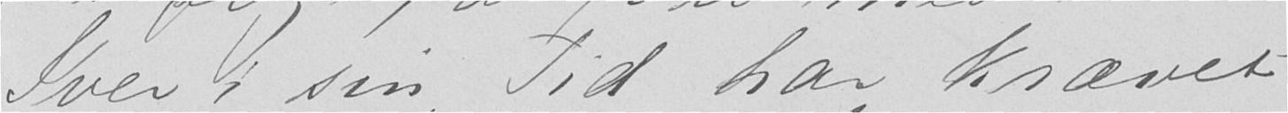Iver i sin Tid har krævet
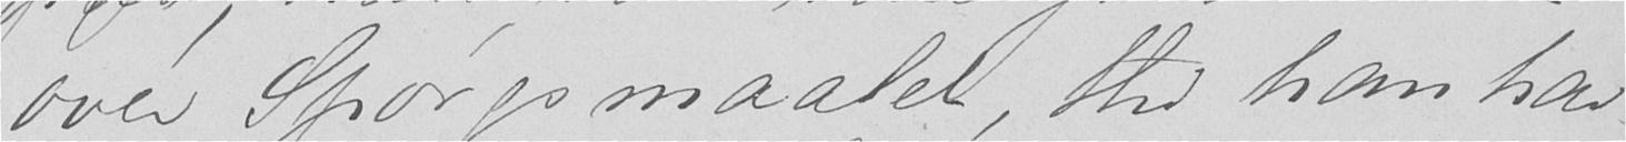over Spørgsmaalet, thi han hav-
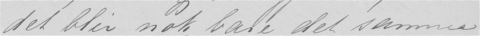det blir nok bare det samme
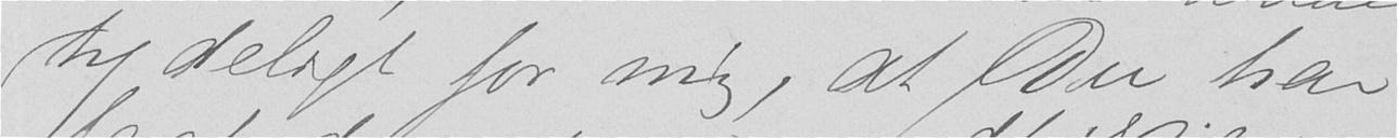tydeligt for mig, at Du har
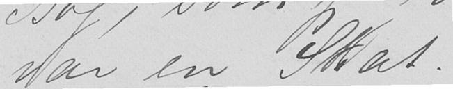var en Skat.
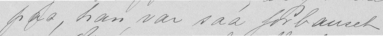paa, han var saa forbauset
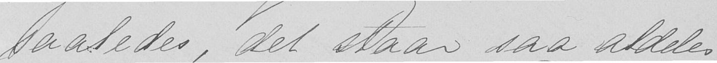saaledes, det staar saa aldeles
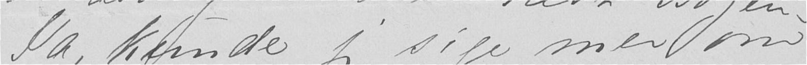Ja, kunde jeg sige mer om
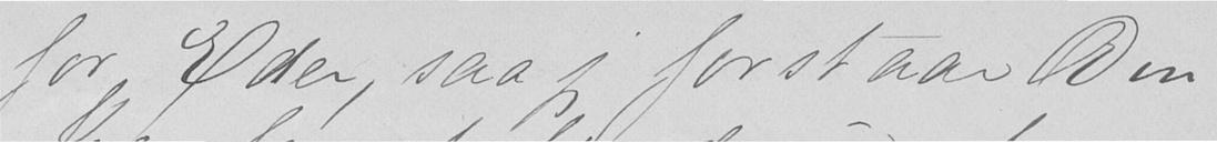for Eder, saa jeg forstaar Din
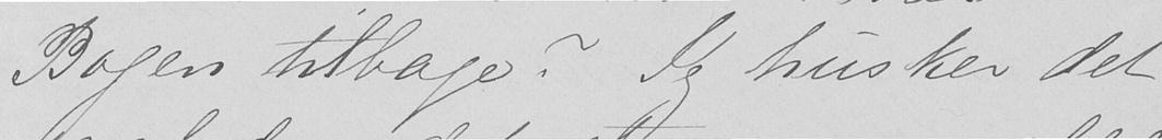Bogen tilbage? Jeg husker det
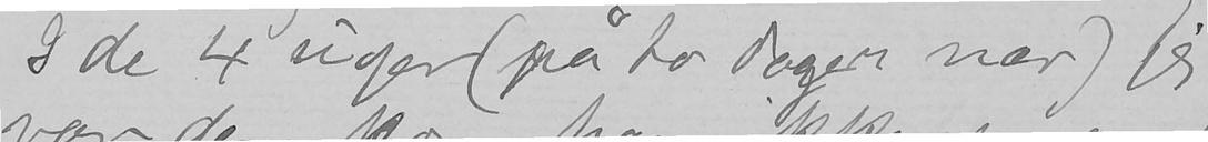I de 4 uger (på to dager nær) jeg
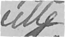celle
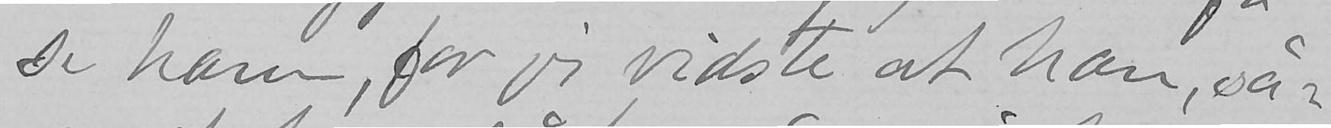se ham, for jeg vidste at han, så-
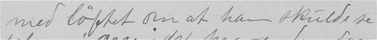med løftet om at han skulde se
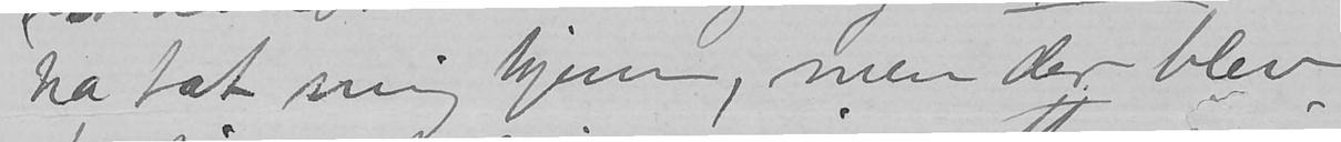ha tat mig hjem, men der blev
